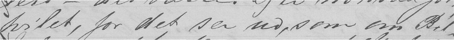tvilet, for det ser ud, som om Bil-
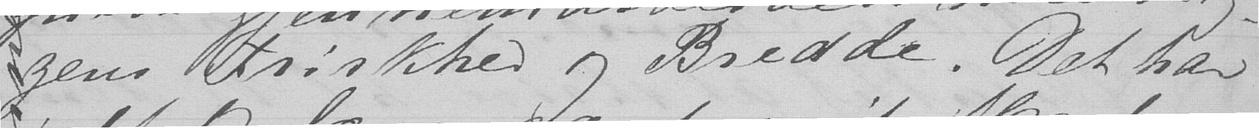zens Friskhed og Bredde. Det har
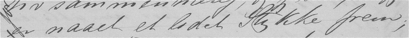er naaet et lidet Stykke frem;
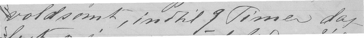voldsomt, indtil 9 Timer dag-
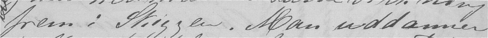frem i Skizzen. Man uddanner
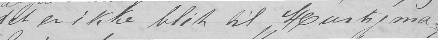det er ikke blit til Hurtigma-
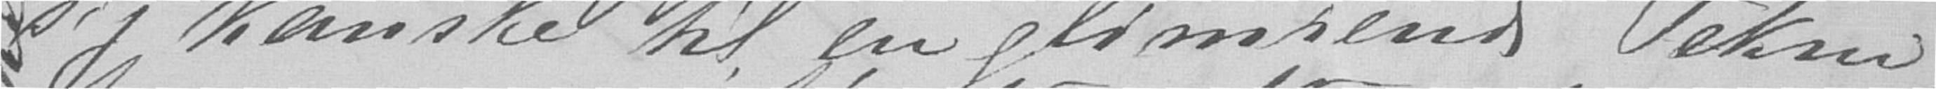sig kanske til en glimrende Tekni-
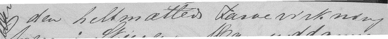og den heltmættede Farvevirkning
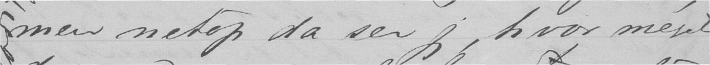men netop da ser jeg hvor meget
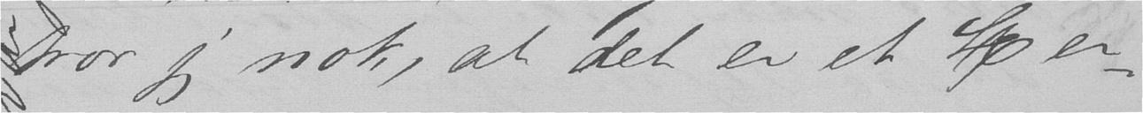tror jeg nok, at det er et Her-
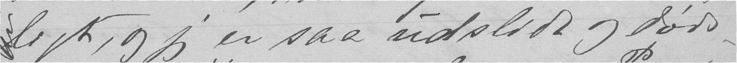ligt, og jeg er saa udslidt og døds-
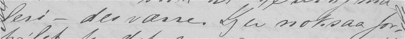leri - desværre. Jeg er noksaa for-
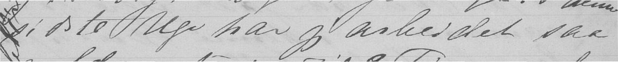sidste Uge har jeg arbeidet saa
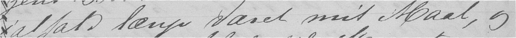ialfald længe været mit Maal, og
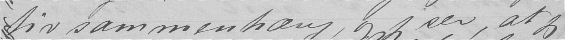tivsammenhæng, og jeg ser, at jeg
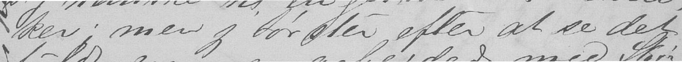ker; men jeg tørster efter at se det
